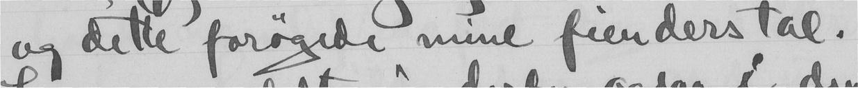og dette forøgede mine fienders tal.
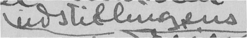udstillingens
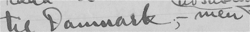til Danmark, - men
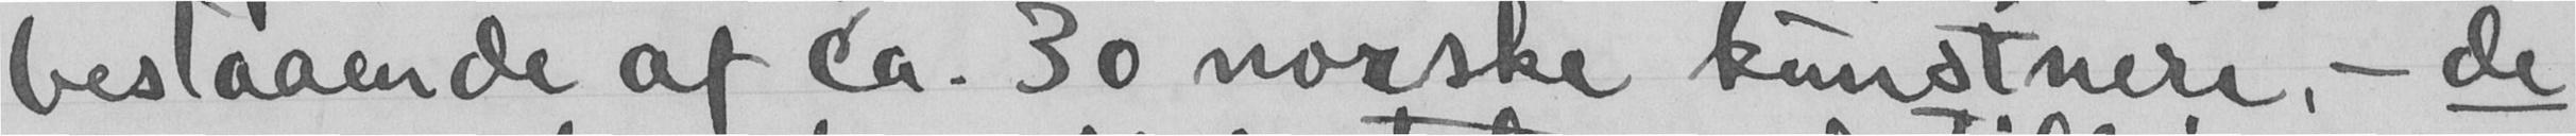bestaaende af ca. 30 norske kunstnere, - de
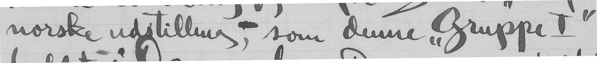norske udstilling, som denne "Gruppe I"
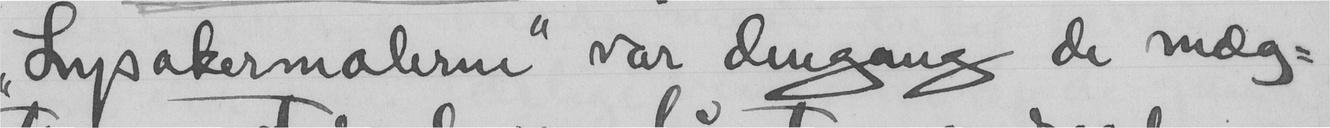"Lysakermalerne" var dengang de mæg-
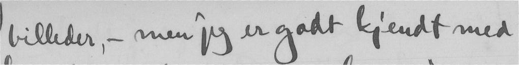billeder, - men jeg er godt kjendt med
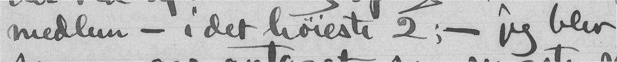medlem - i det høieste 2; - jeg blev
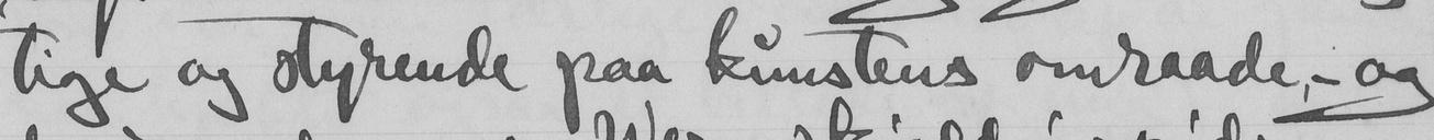tige og styrende paa kunstens omraade, - og
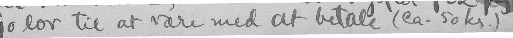jo lov til at være med at betale (ca. 50 kr.)
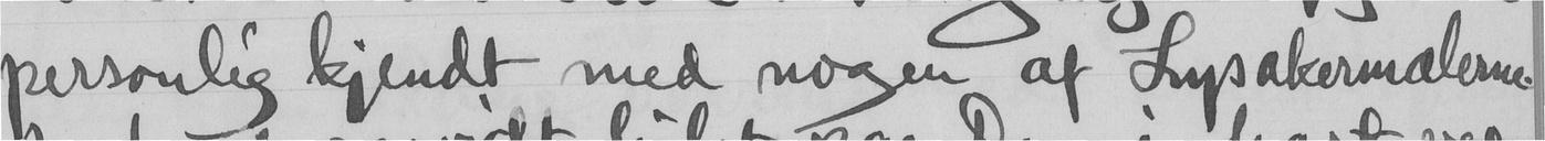personlig kjendt med nogen af Lysakermalerne.
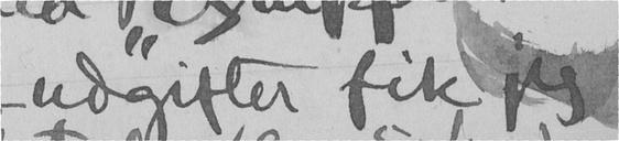udgifter fik jeg
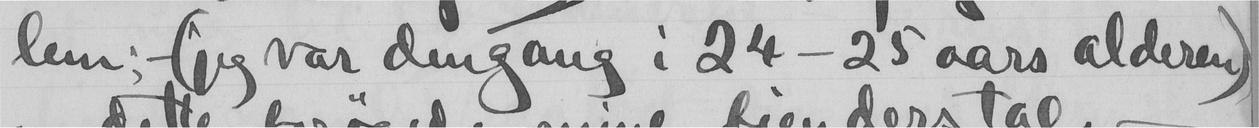lem;- (jeg var dengang i 24 - 25 aars alderen)
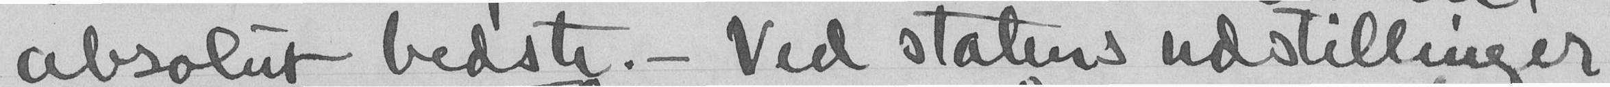absolut bedste. - Ved statens udstillinger
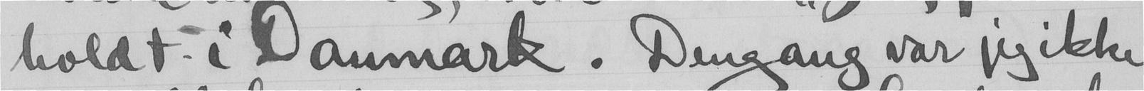holdt i Danmark. Dengang var jeg ikke
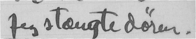Jeg stængte døren.
